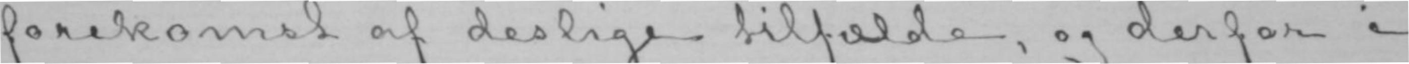forekomst af deslige tilfælde, og derfor i
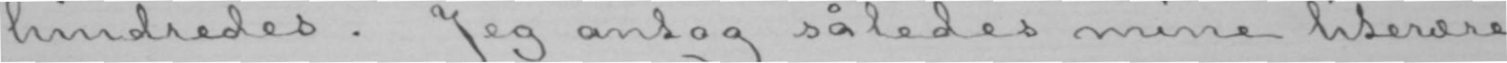hindredes. Jeg antog således mine literære
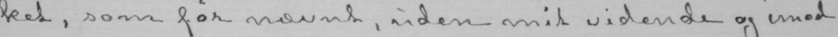ket, som før nævnt, uden mit vidende og imod
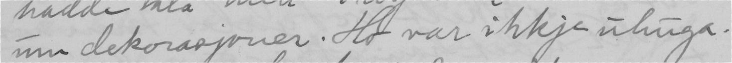um dekorasjoner. Ho var ikkje uhuga.
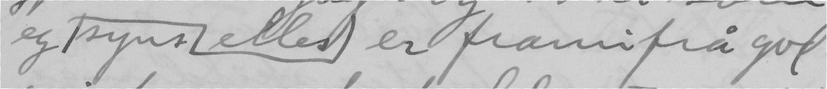eg elles syns er framifrå god
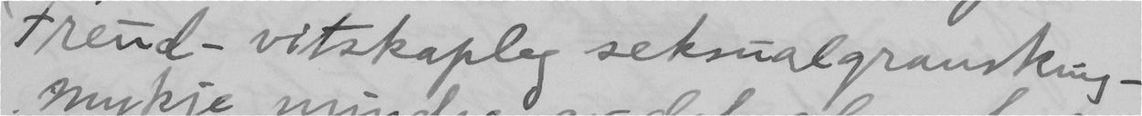Freud-vitskapleg seksualgransking –
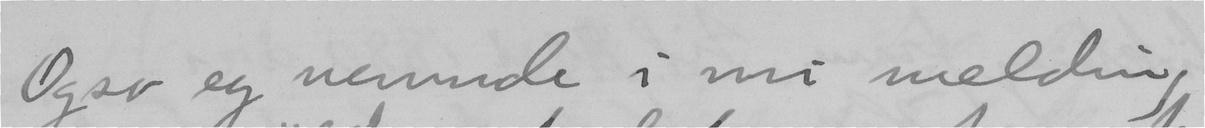Ogso eg nemde i mi melding
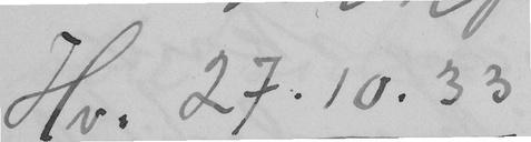Hv. 27.10.33
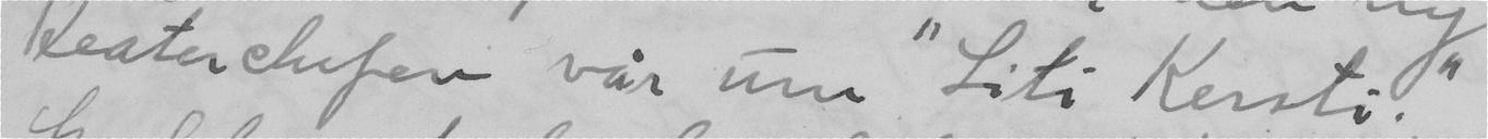teaterchefen vår um "Liti Kersti."
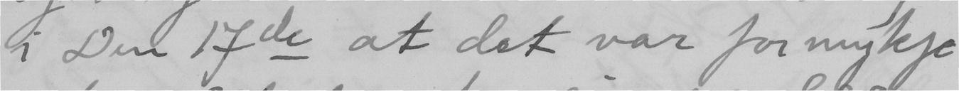i Den 17de at det var for mykje
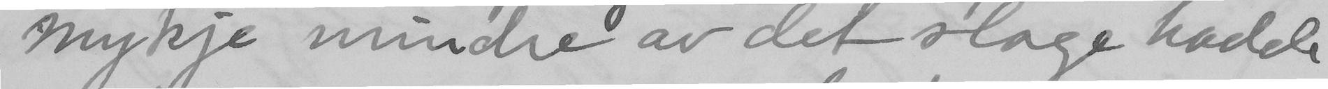mykje mindre av det slage hadde
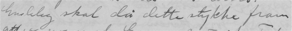Endeleg skal då dette stykke fram
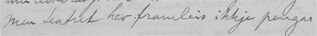Men teatret hev framleis ikkje pengar
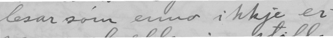lesar som enno ikkje er
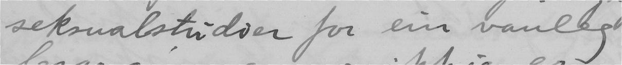seksualstudier for ein vanleg
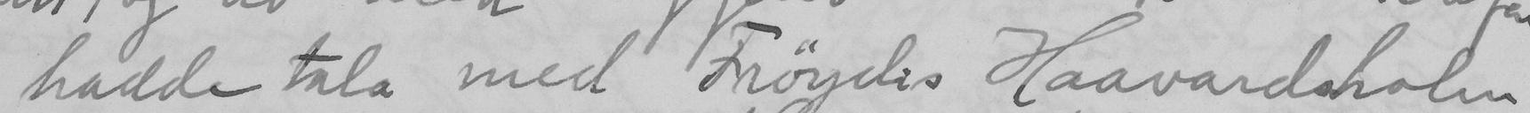hadde tala med Fröydis Haavardsholm
